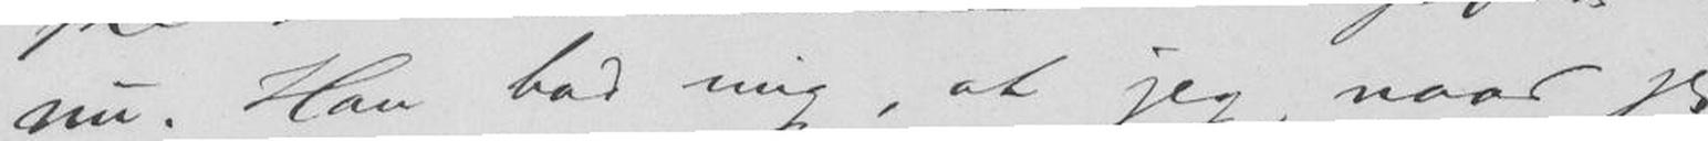nu. Han bad mig, at jeg, naar jeg
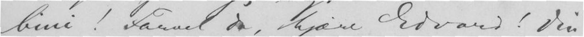bini! Farvel da, kjære Edvard! Din
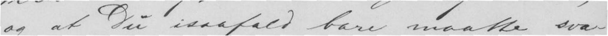og at Du isaafald bare maatte sva-
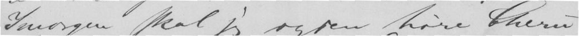Imorgen skal jeg og...en høre Cheru-
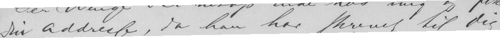Din Adresse, da han har skrevet til Dig
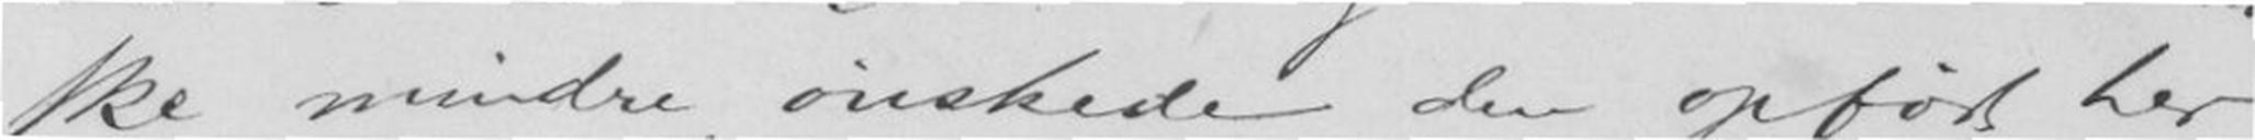ske mindre ønskede den opført her
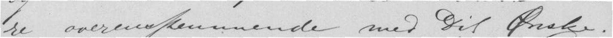re overensstemmende med Dit Ønske.
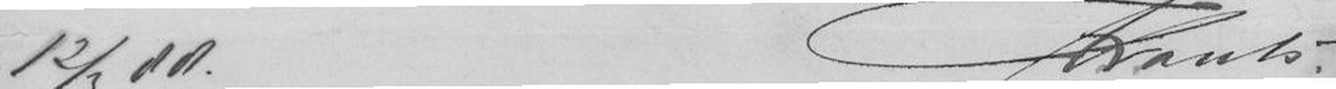12/3 88 Frants.
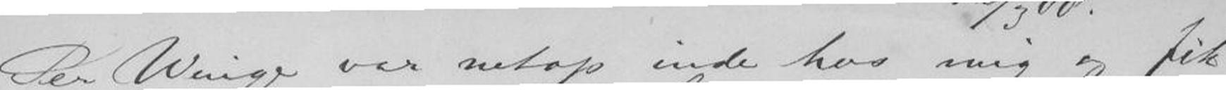Per Winge var netop inde hos mig og fik
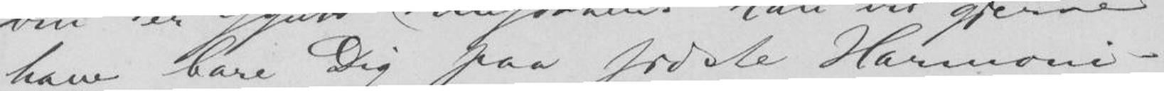have bare Dig paa sidste Harmoni-
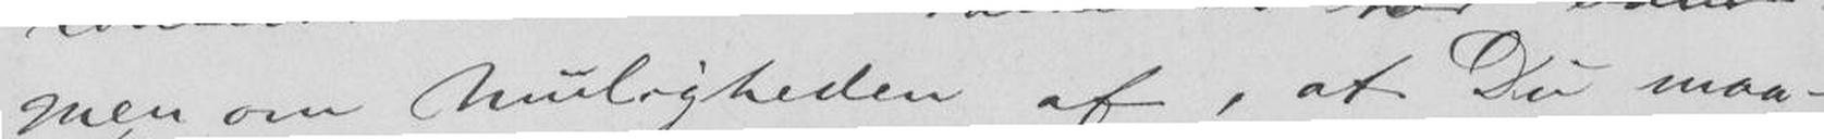men om Muligheden af, at Du maa-
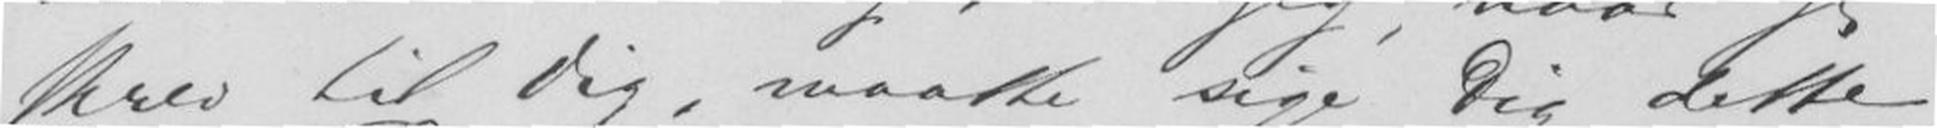skrev til Dig, maatte sige Dig dette,
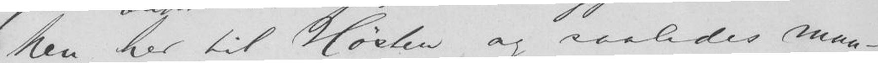ken her til Høsten og saaledes maa-
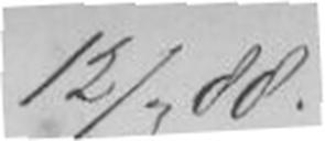12/3 88
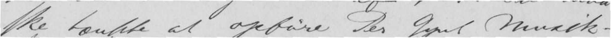ske tænke at opføre Per Gynt Musik-
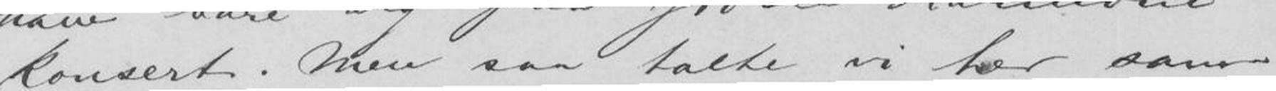konsert. Men saa talte vi her sam-
